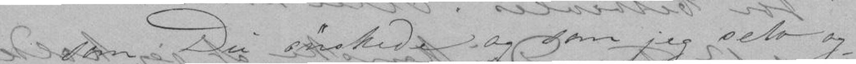som Du ønskede og som jeg selv og-
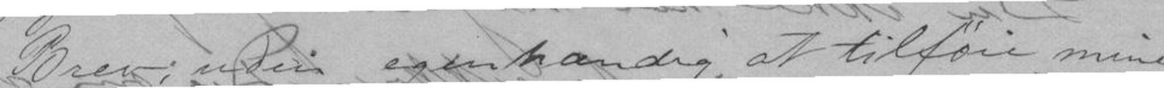Brev; uden egenhændig at tilføie mine
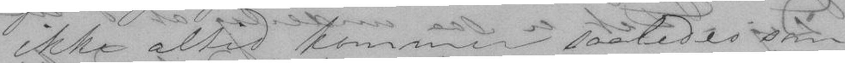ikke altid kommer saaledes som
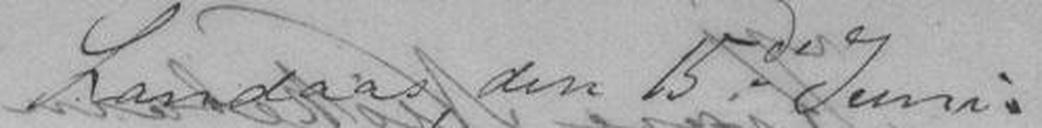Landaas, den 15de Juni.
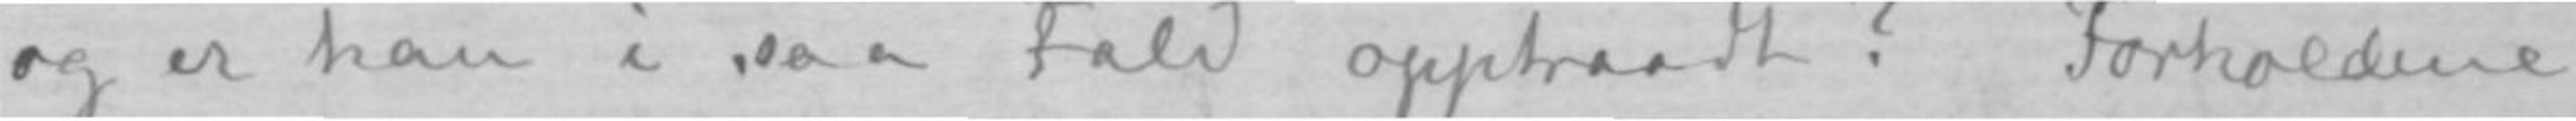og er han i saa Fald opptraadt? Forholdene
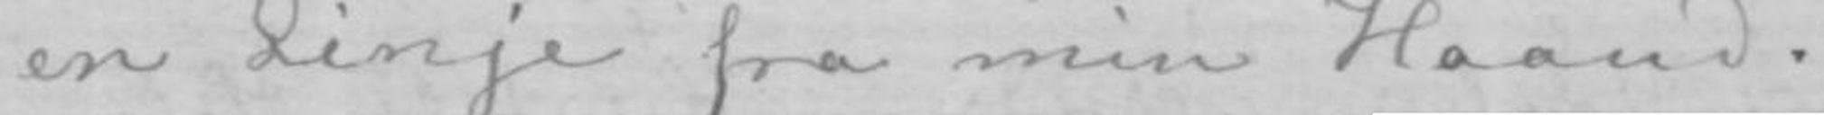en Linje fra min Haand.
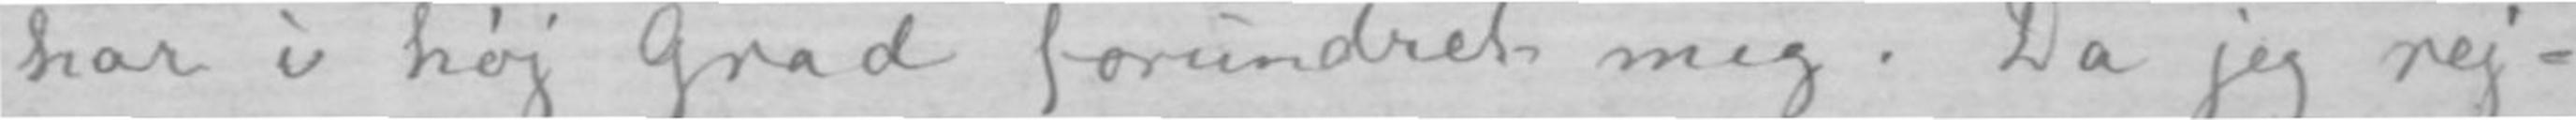har i høj Grad forundret mig. Da jeg rej-
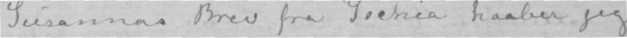Susannas Brev fra Ischia haaber jeg
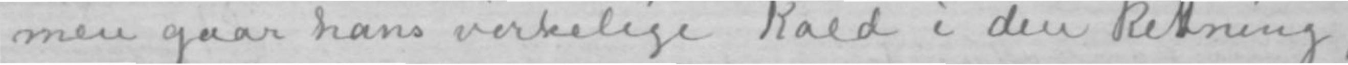men gaar hans virkelige Kald i den Rettning,
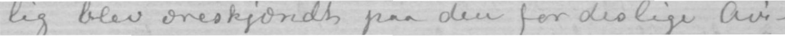lig blev æreskjændt paa den for deslige Avi-
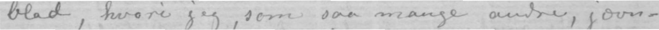blad, hvori jeg, som saa mange andre, jævn-
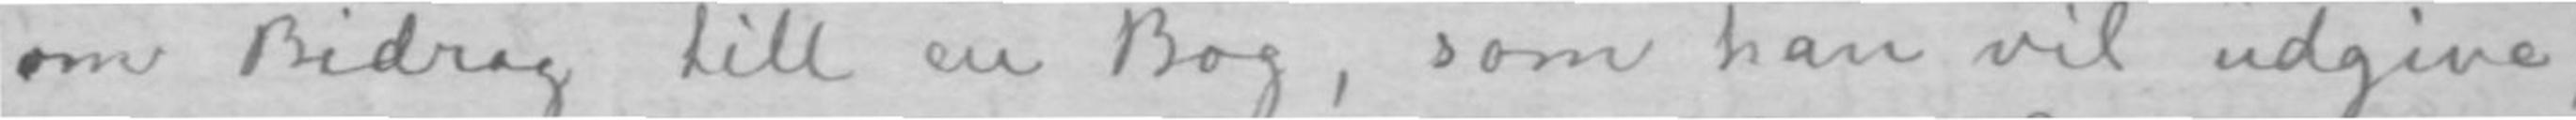om Bidrag till en Bog, som han vil udgive,
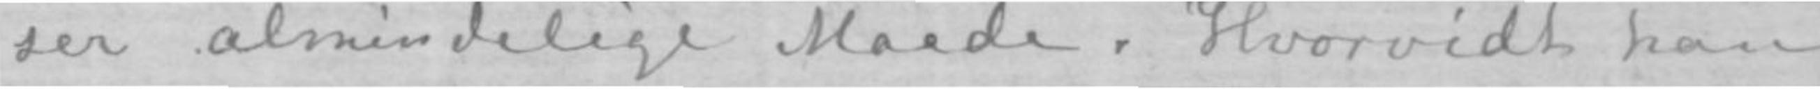ser almindelige Maade. Hvorvidt han
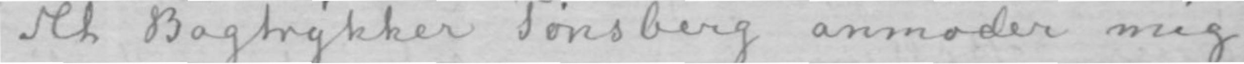At Bogtrykker Tønsberg anmoder mig
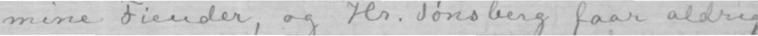mine Fiender, og Hr. Tønsberg faar aldrig
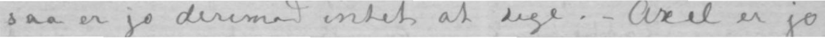saa er jo derimod intet at sige.- Axel er jo
